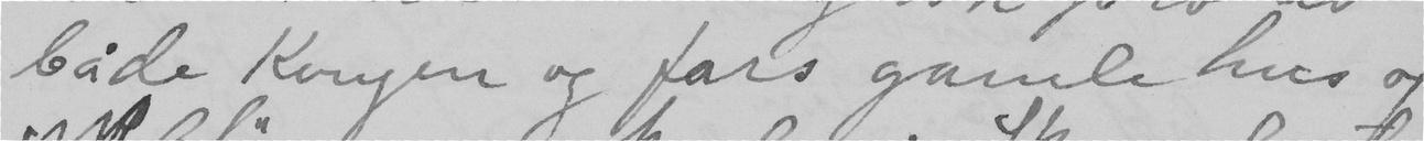både Kongen og fars gamle hus og
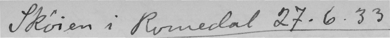Sköien i Romedal 27.6.33
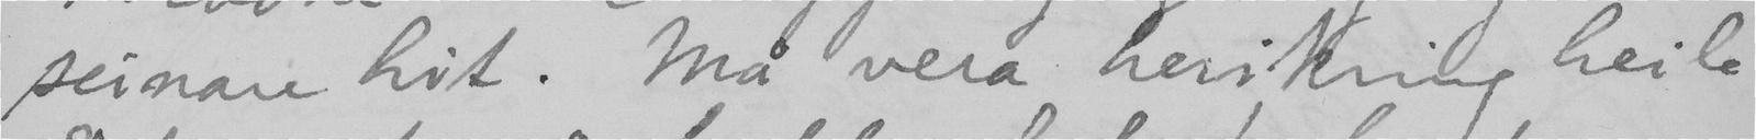seinare hit. Må vera herikring heile
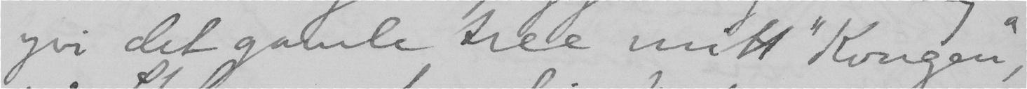yvi det gamle tree mitt "Kongen",
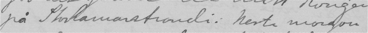på Stormanstrandi. Neste morgon
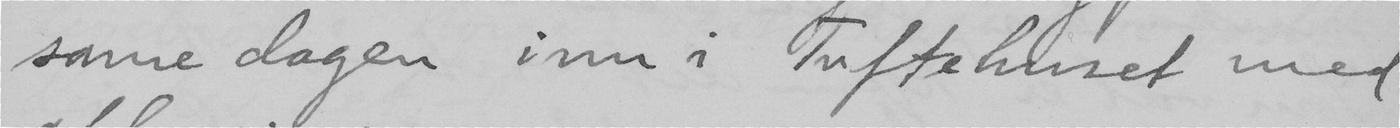same dagen inn i Tuftehuset med
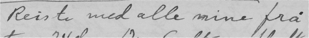Reiste med alle mine frå
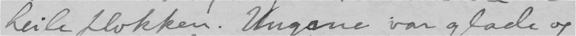heile flokken. Ungane var glade og
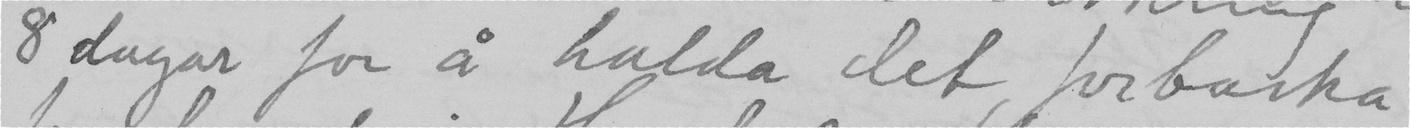8 dagar for å halda det forbaska
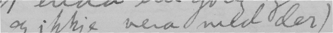eg ikkje vera med der)
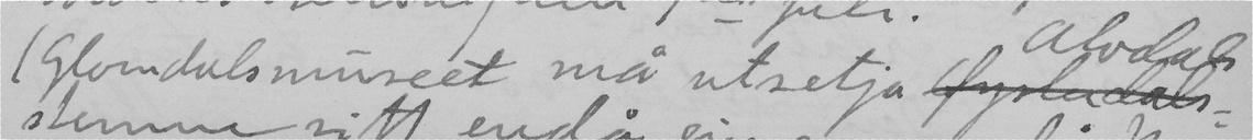(Glomdalsmuseet må utsetja Alvdals-
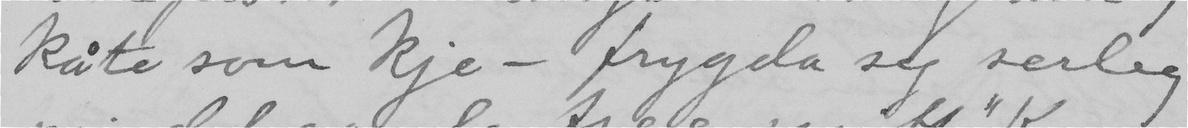kåte som kje – frygda sig serleg
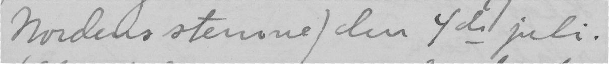Nordens stemne) den 4de juli.
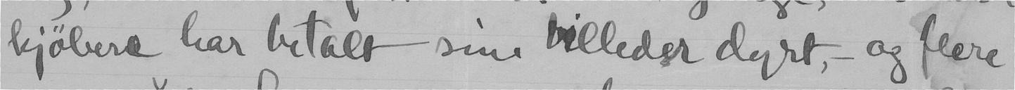kjøberd har betalt sine billeder dyrt, - og flere
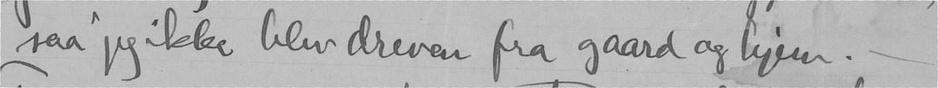saa jeg ikke blev dreven fra gaard og hjem. -
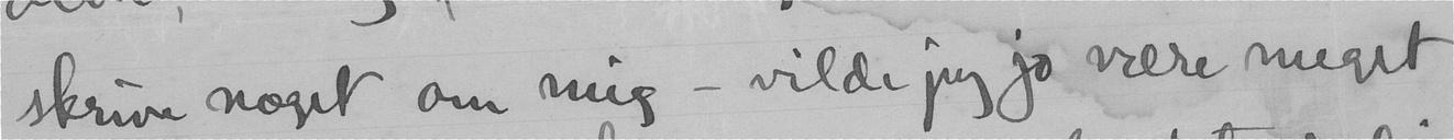skrive noget om mig - vilde jeg jo være meget
